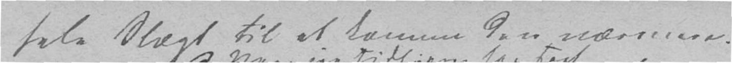hele Slægt til at komme den nærmere.
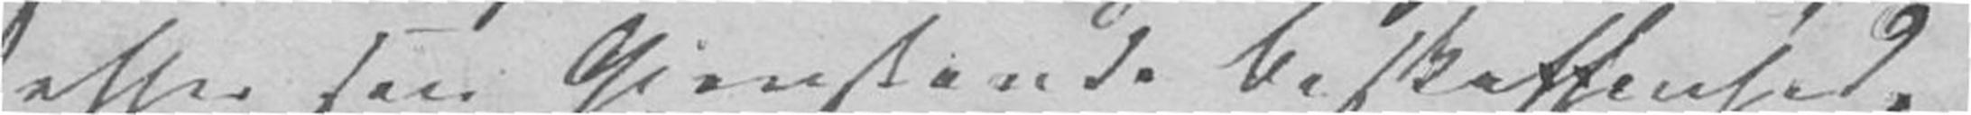efter sin Gjenstands Beskaffenhed,
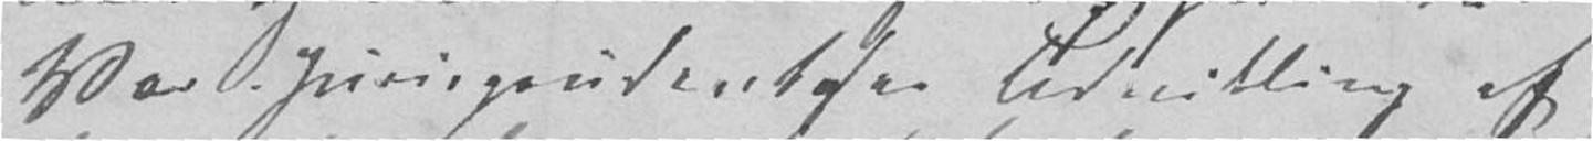Var Jurisprudentens Udvikling af
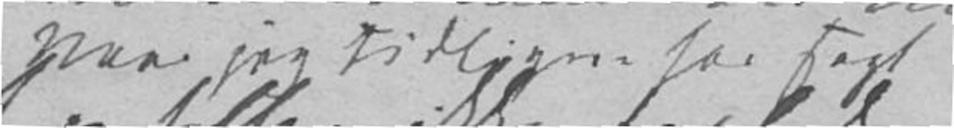paa jeg tidligere har sagt
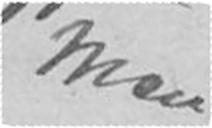Man
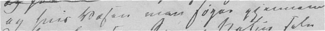og hvis Væsen man søger gjennem
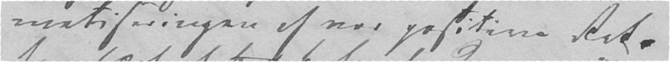ematiseringen af vor positive Ret.
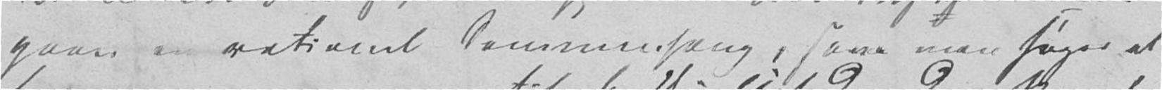gaar en rationel Sammenhæng, som man søger at
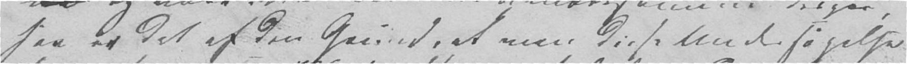saa er det af den Grund, at men disse Undersøgelser
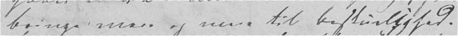bringe mere og mere til Beskuelighed.
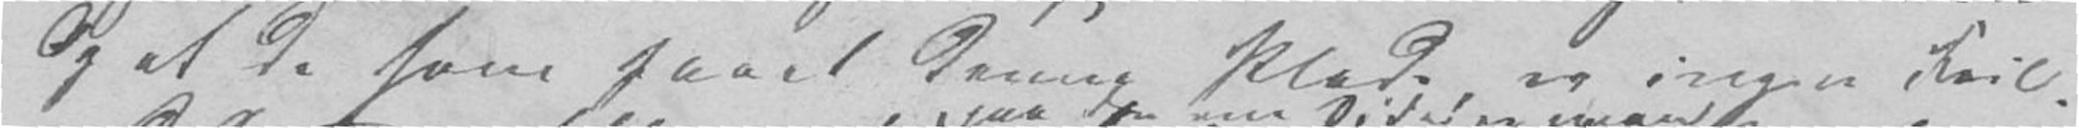Og at de have faaet denne Plads, er ingen Feil
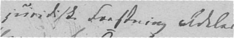juridiske Forskning aldeles
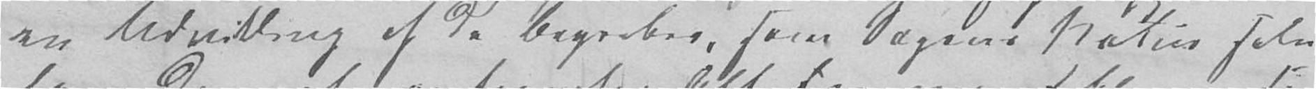en Udvikling af de Begreber, som Sagens Natur selv
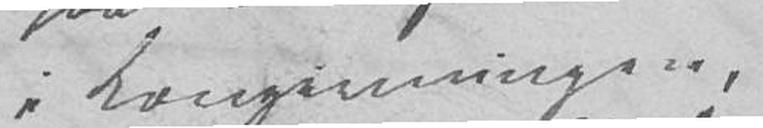i Lovgivningen,
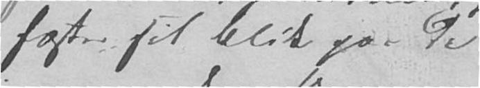fæster sit Blik paa de
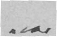vor
